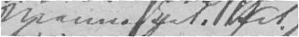Menneskerettighed
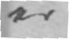er
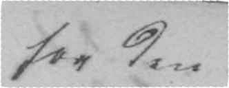for den
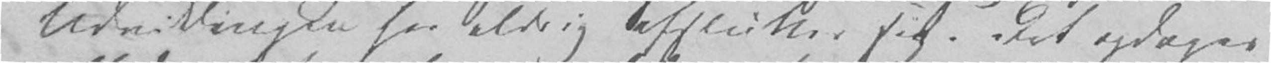Udviklingen her aldrig afslutter sig. Det opdages
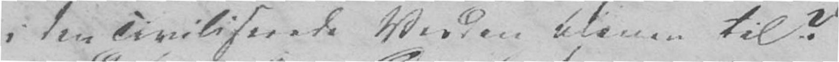i den civiliserede Verden bleven til?
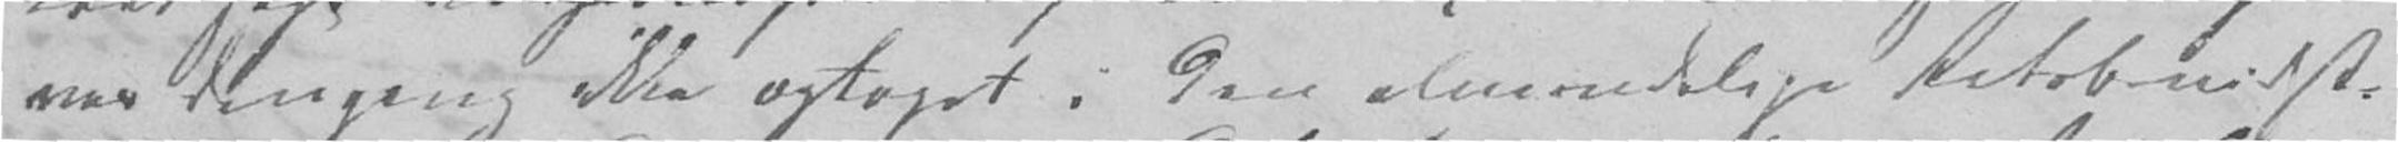var dengang ikke optaget i den almindelige Retsbevidst-
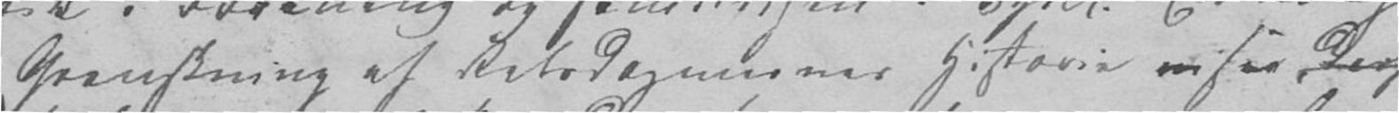Granskning af Retsdogmernes Historie viser,
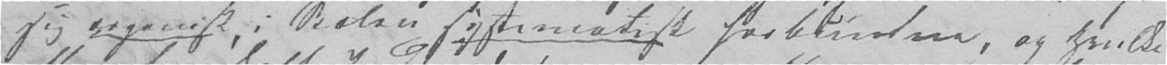sig organisk, i Staten systematisk forbundne, og hvilke
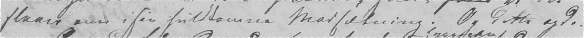slaaer om i sin fuldkomne Modsætning. Og dette opda-
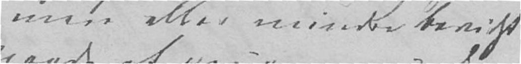mere eller mindre bevidst
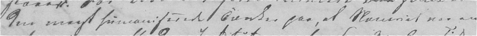den meest humaniserede Tænker paa, at Slaveriet var en
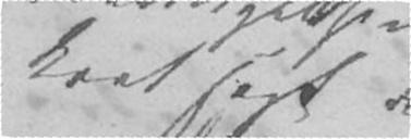kort sagt
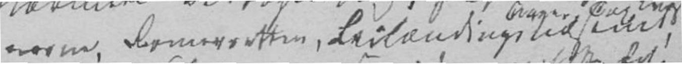nerne, Romerretten, Leilændingsvæsenet
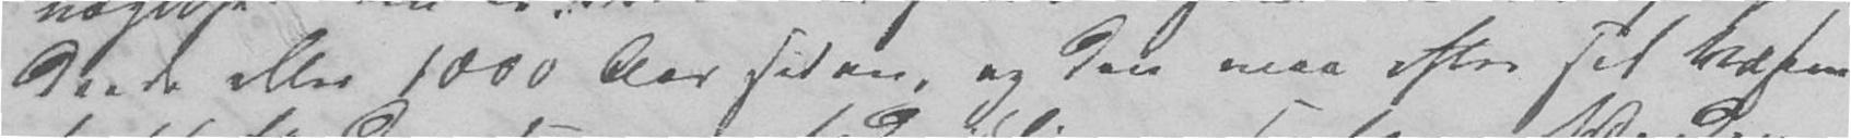drede eller 1000 Aar siden, og den maa efter sit Væsen
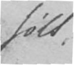følt,
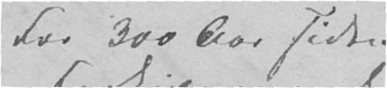For 300 Aar siden
